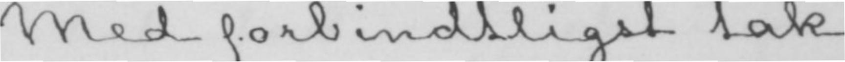Med forbindtligst tak
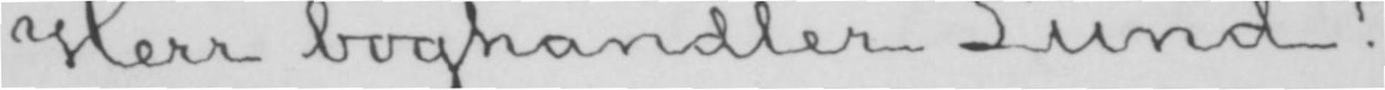Herr boghandler Lund!
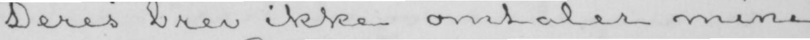Deres brev ikke omtaler mine
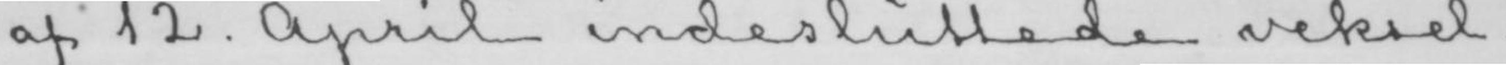af 12. April indesluttede veksel.
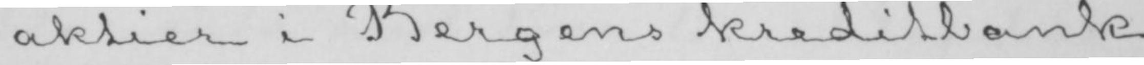aktier i Bergens kreditbank,
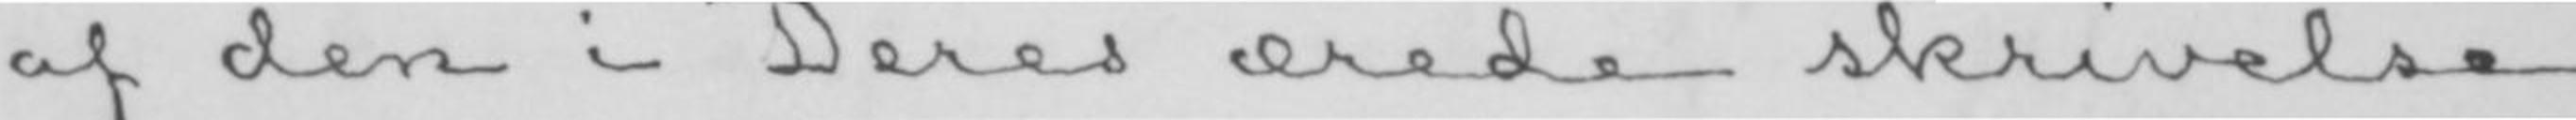af den i Deres ærede skrivelse
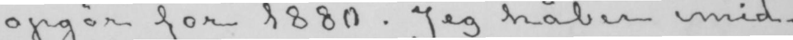opgør for 1880. Jeg håber imid-
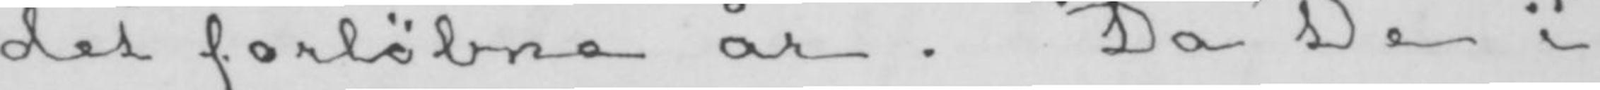det forløbne år. Da De i
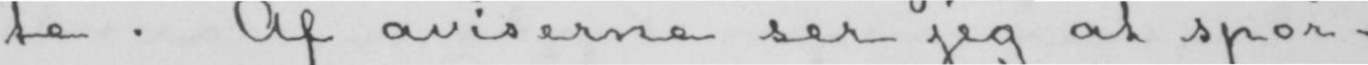te. Af aviserne ser jeg at spor-
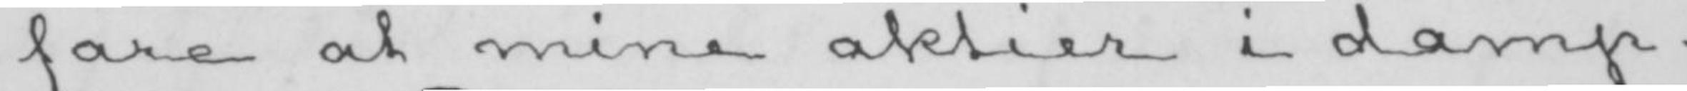fare at mine aktier i damp-
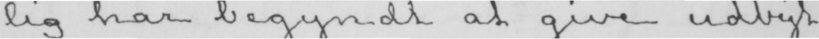lig har begyndt at give udbyt-
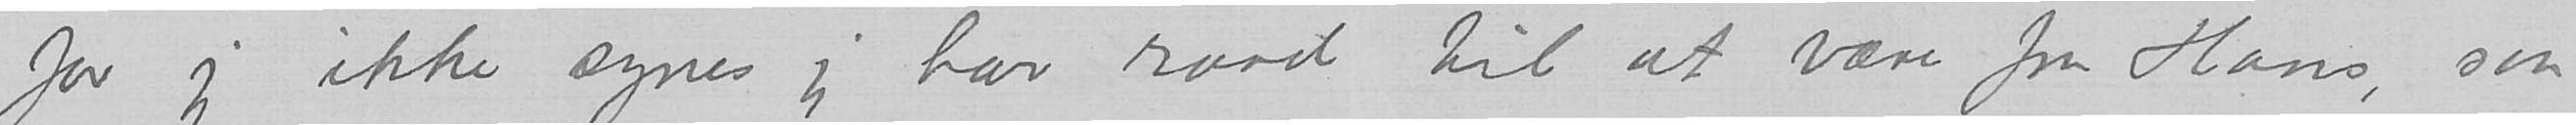for jeg ikke synes jeg har raad til at være fra Hans, saa
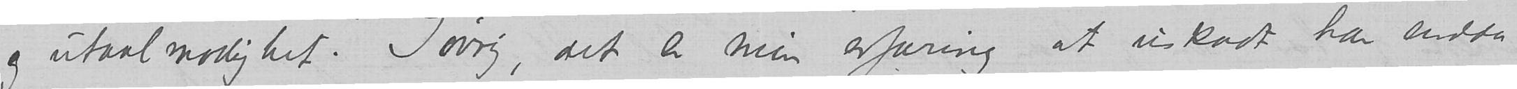og utaalmodighet. Iøvrig, det er min erfaring at uskadt har endda
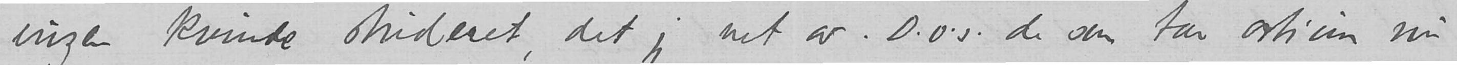ingen kvinde studeret, det jeg vet av. D.v.s. de som tar artium nu
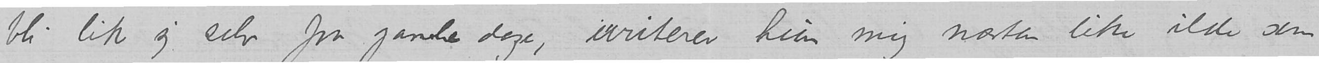bli lik sig selv fra gamle dager, irriterer hun mig næsten like ilde som
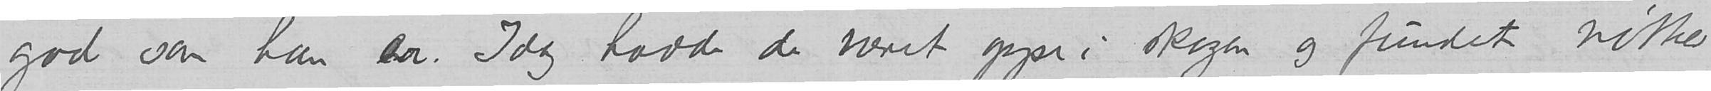god som han er. Idag hadde de været oppe i skogen og fundet nøtter,
